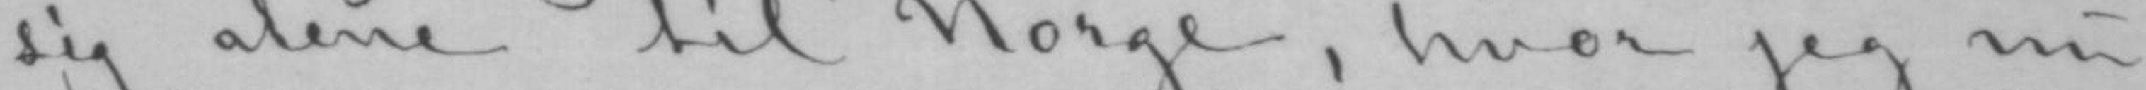sig alene til Norge, hvor jeg nu
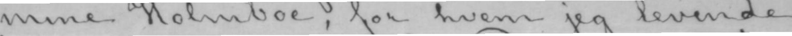mine Holmboe, for hvem jeg levende
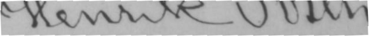Henrik Ibsen.
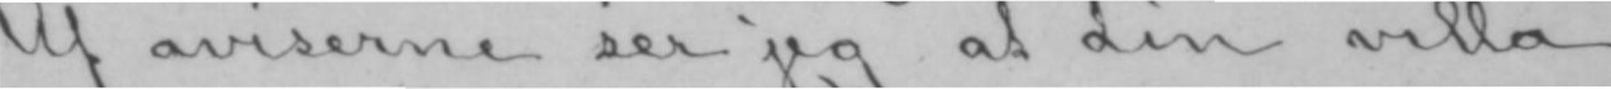Af aviserne ser jeg at din villa
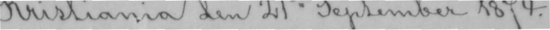Kristiania den 21de September 1874.
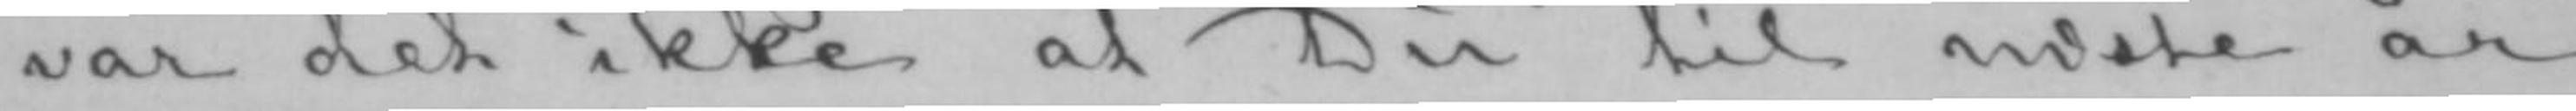var det ikke at Du til næste år
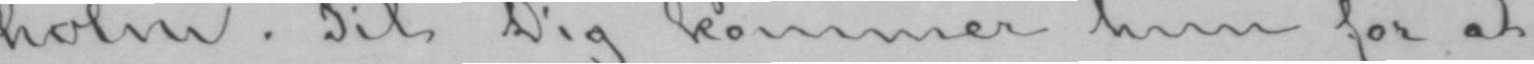holm. Til Dig kommer hun for at
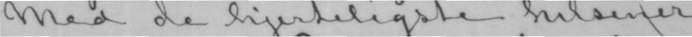Med de hjerteligste hilsener
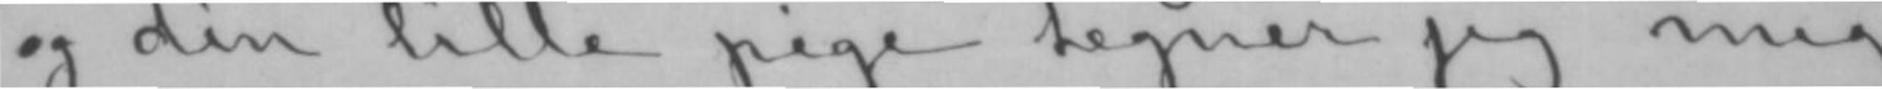og din lille pige tegner jeg mig
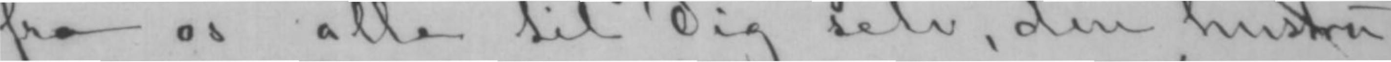fra os alle til Dig selv, din hustru
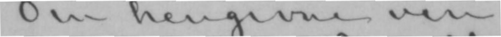Din hengivne ven
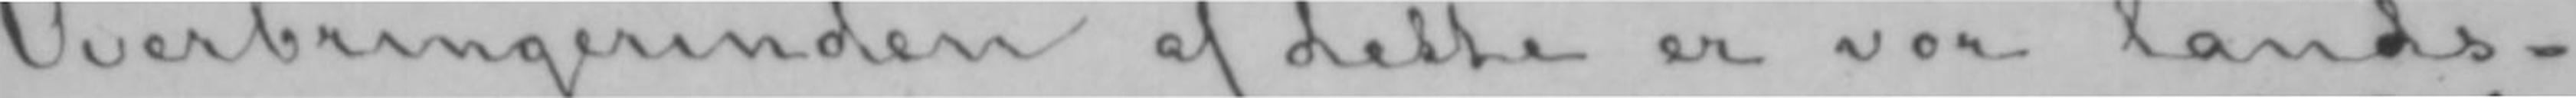Overbringerinden af dette er vor lands-
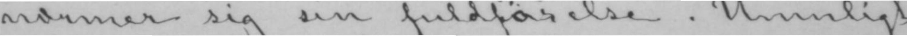nærmer sig sin fuldførelse. Umuligt
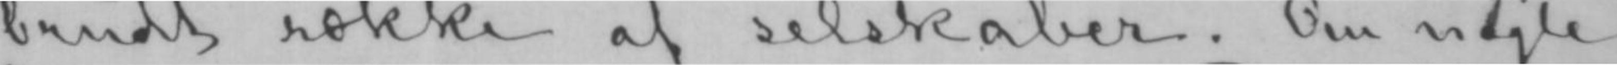brudt række af selskaber. Om nogle
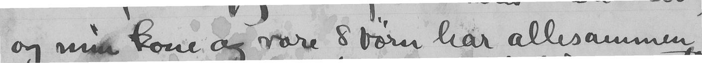og min kone og være 3 børn har allesammen
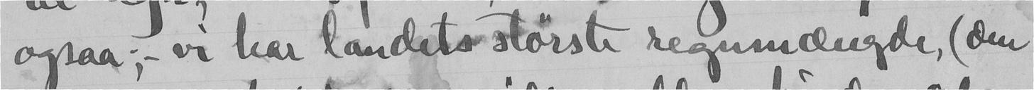ogsaa; - vi har landets største regnmængde, (den
